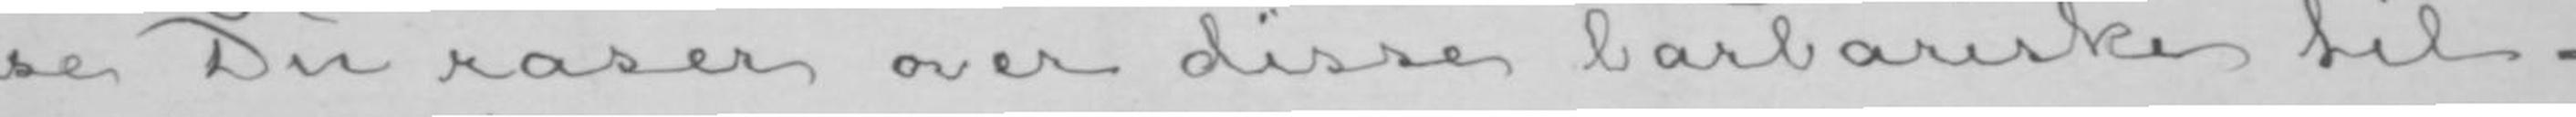se Du raser over disse barbariske til-
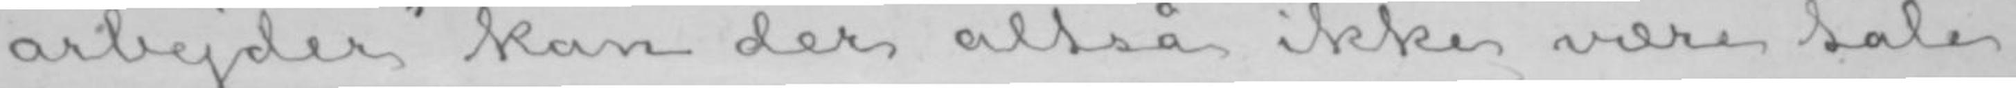arbejder» kan der altså ikke være tale
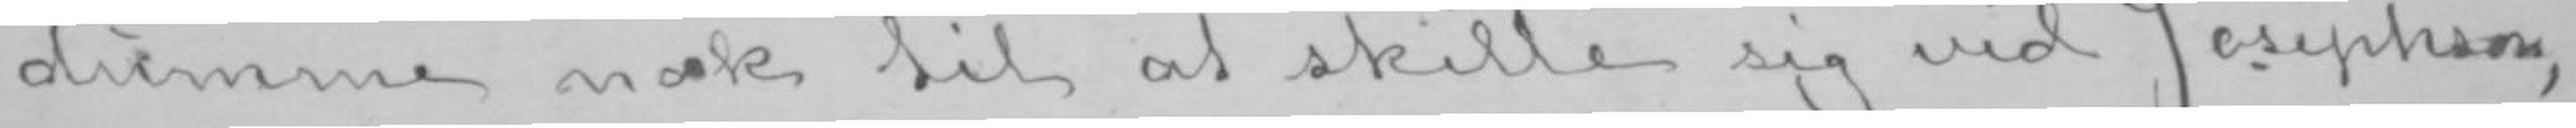dumme nok til at skille sig vid Josephson,
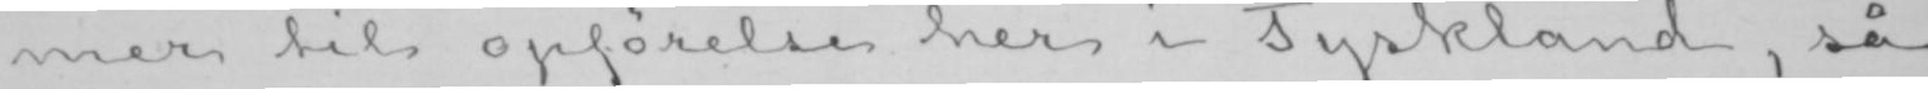mer til opførelse her i Tyskland, så
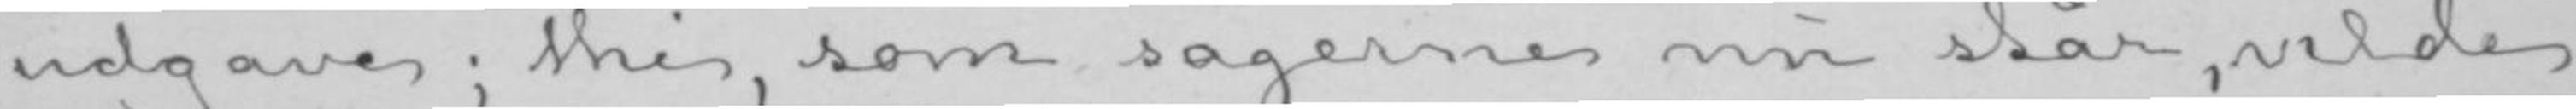udgave; thi, som sagerne nu står, vilde
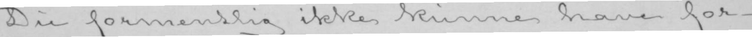Du formentlig ikke kunne have for-
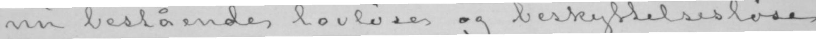nu bestående lovløse og beskyttelsesløse
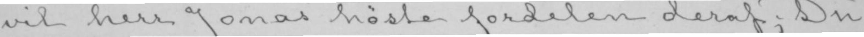vil herr Jonas høste fordelen deraf; Du
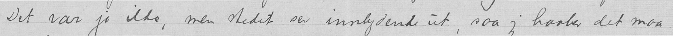Det var jo ilde, men stedet ser innbydende ut, saa jeg haaber det maa
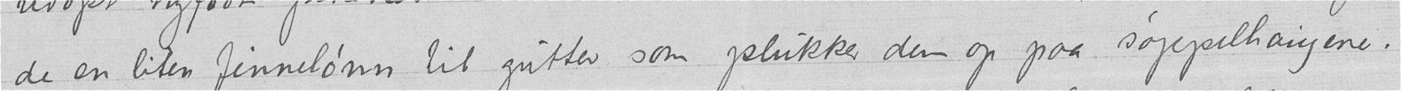de en liten finnelønn til gutter som plukker dem op paa søppelhaugene.
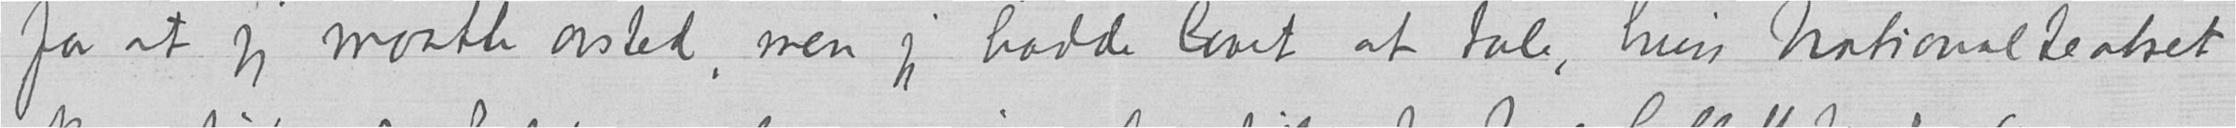for at jeg maatte avsted, men jeg hadde lovet at tale, hvis Nationalteatret
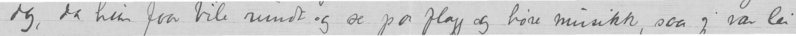dag, da hun faar bile rundt og se paa flagg og høre musikk, saa jeg var lei
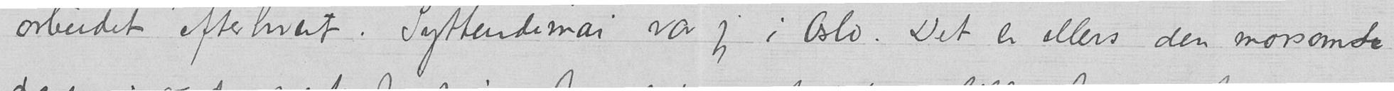arbeidet efterhvert. Syttendemai var jeg i Oslo. Det er ellers den morsomste
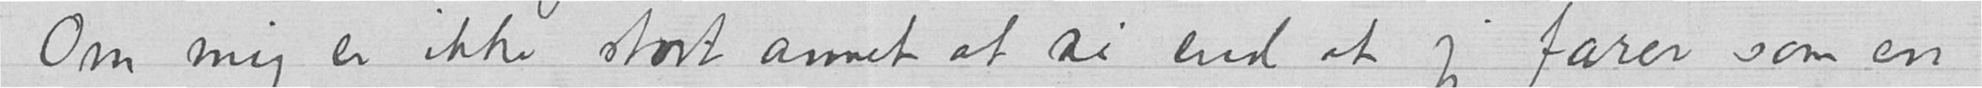Om mig er ikke stort annet at si end at jeg farer som en
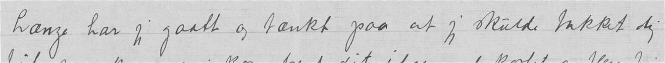Længe har jeg gaatt og tænkt paa at jeg skulde takket dig
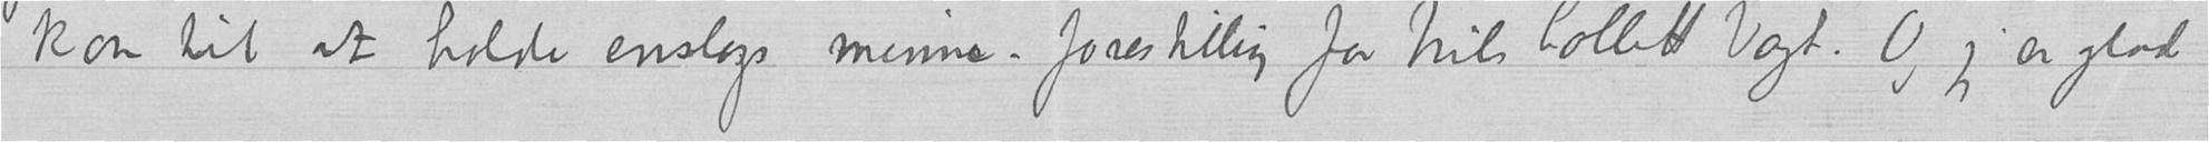kom til at holde enslags minne-forestilling for Nils Collett Vogt. Og jeg er glad
